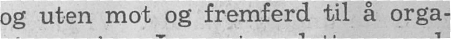og uten mot og fremferd til å orga-
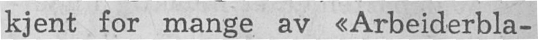kjent for mange av "Arbeiderbla-
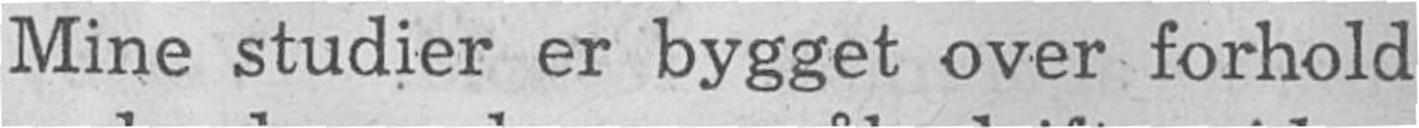Mine studier er bygget over forhold
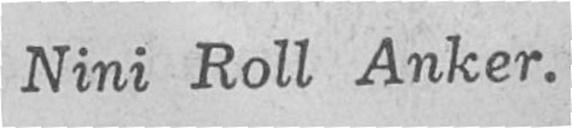Nini Roll Anker.
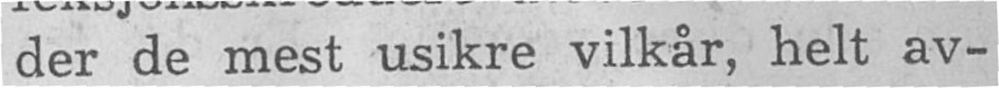der de mest usikre vilkår, helt av
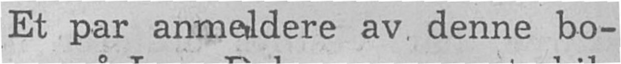Et par anmeldere av denne bo-
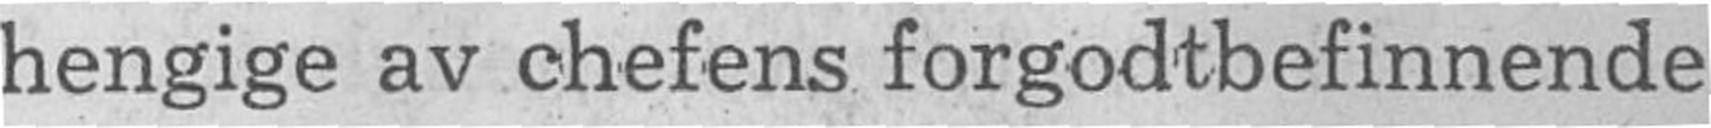hengige av chefens forgodtbefinnende
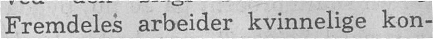Fremdeles arbeider kvinnelige kon-
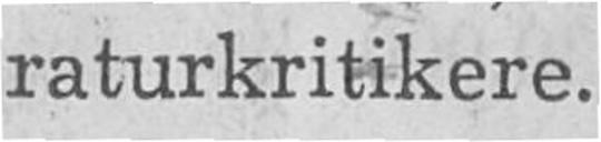raturkritikere.
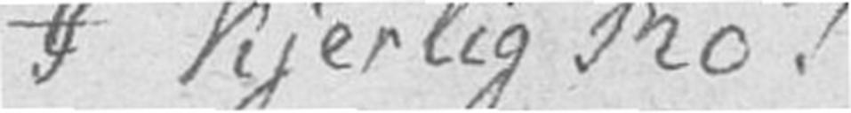I kjerlig Ro!
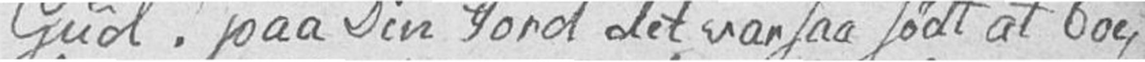Gud! paa Din Jord det var saa sødt at boe,
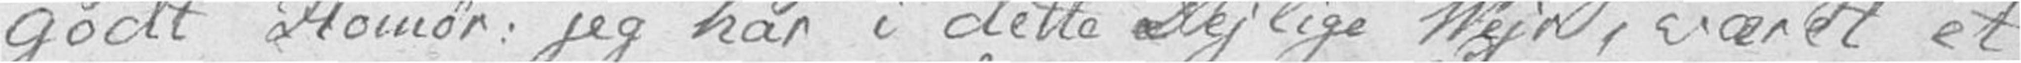godt Homør: jeg har i dette Dejlige Vejr, været et
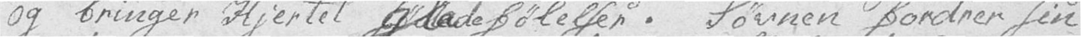og bringer Hjertet glade følelser. Søvnen fordrer sin
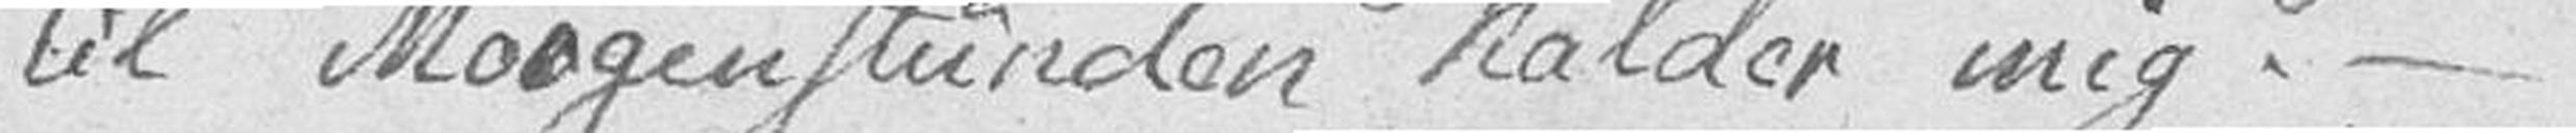til Morgenstunden kalder mig. –
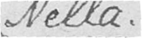Nella
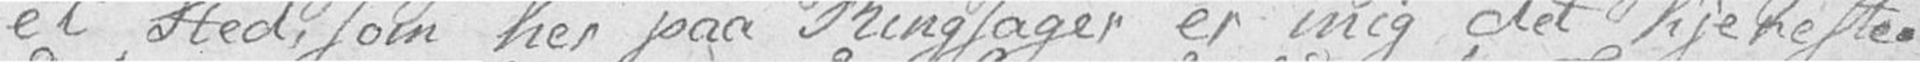et Sted, som her paa Ringsager er mig det kjereste.
